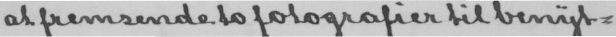at fremsende to fotografier til benyt-
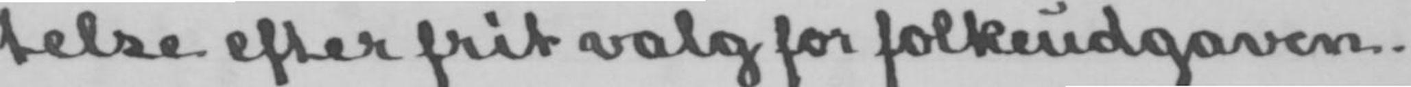telse efter frit valg for folkeudgaven.
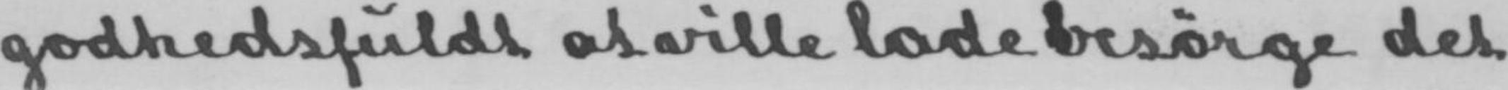godhedsfuldt at ville lade besørge det
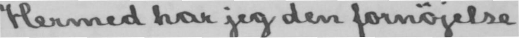Hermed har jeg den fornøjelse
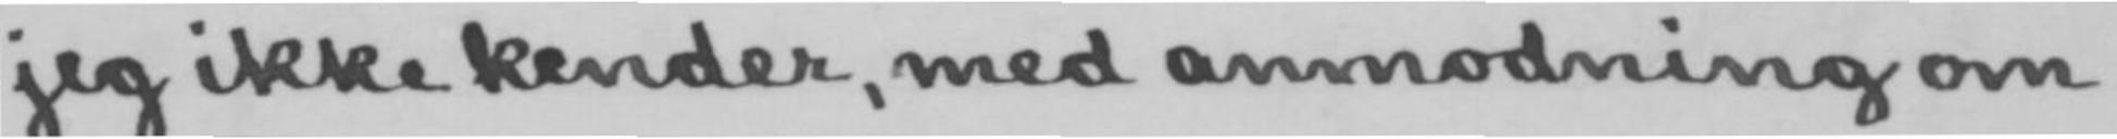jeg ikke kender, med anmodning om
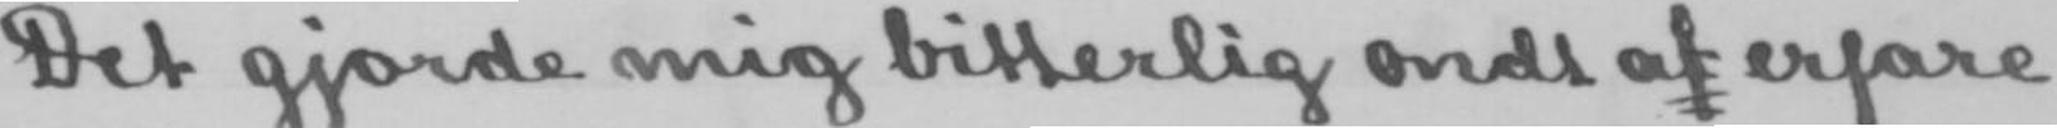Det gjorde mig bitterlig ondt at erfare
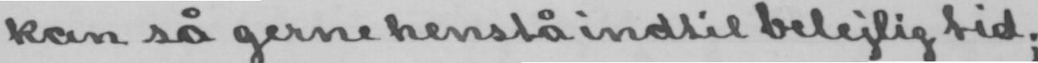kan så gerne henstå indtil belejlig tid;
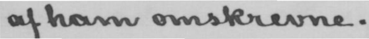af ham omskrevne.
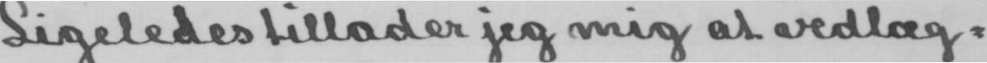Ligeledes tillader jeg mig at vedlæg-
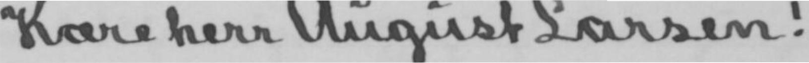Kære herr August Larsen!
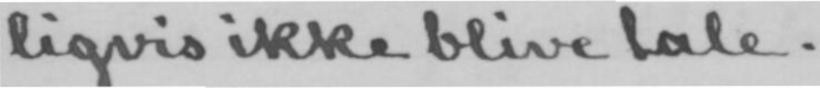ligvis ikke blive tale.
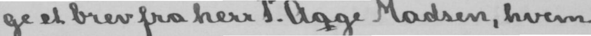ge et brev fra herr P. Aage Madsen, hvem
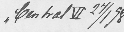"Central VI 27/1 98.
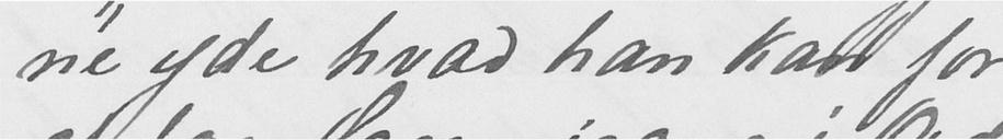ne yde hvad han kan for
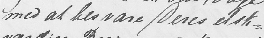med at besvare Deres elsk-
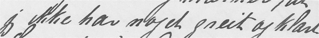jeg ikke har noget greit og klart
